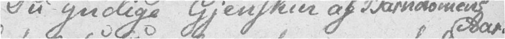Du yndige Gjenskin af Barndommens Aar!
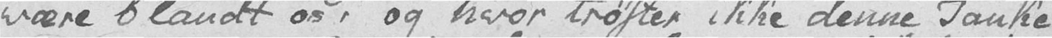være blandt os, og hvor trøster ikke denne Tanke
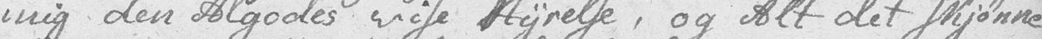mig den Algodes vise Styrelse, og Alt det skjønne
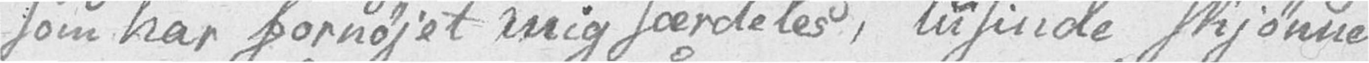som har fornøjet mig særdeles, tusinde skjønne
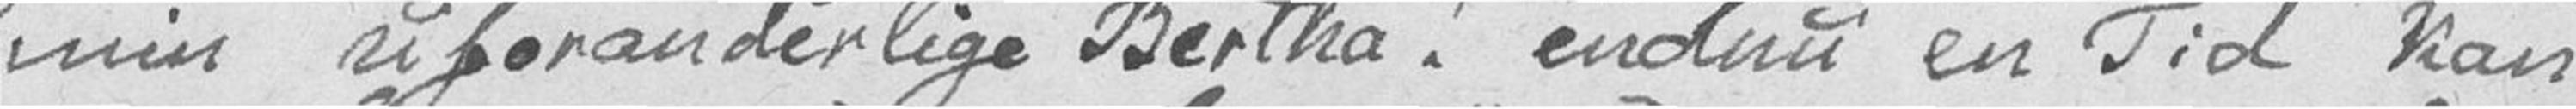min uforanderlige Bertha! endnu en Tid kan
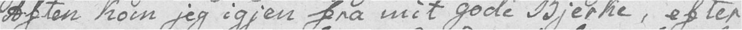Aften kom jeg igjen fra mit gode Bjerke, efter
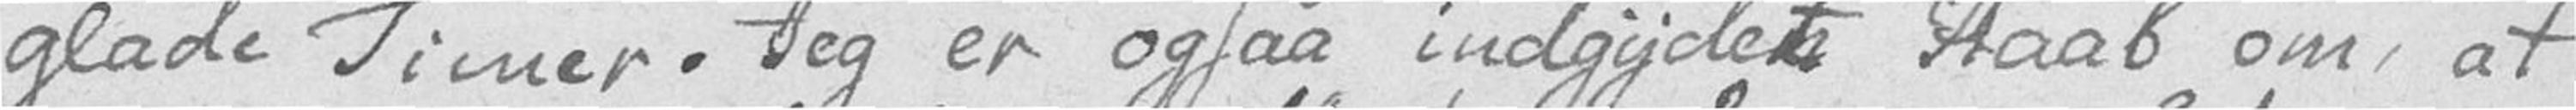glade Timer. Jeg er ogsaa indgydet Haab om, at
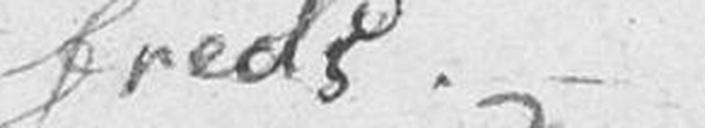freds. –
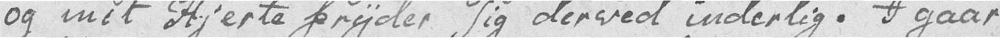og mit Hjerte fryder sig derved inderlig. I gaar
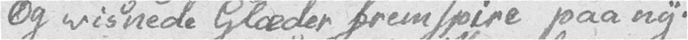Og visnede Glæder fremspire paa ny.
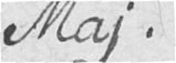Maj.
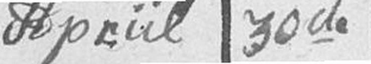Apriil 30de
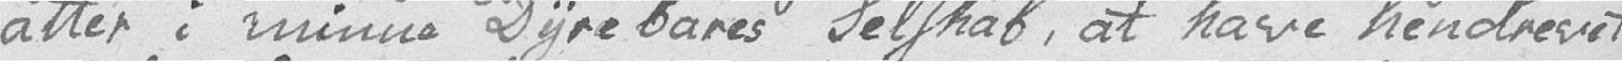atter i minne Dyrebares Selskab, at have hendrevet
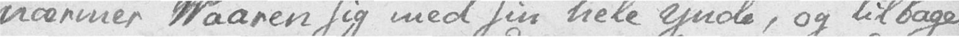nærmer Vaaren sig med sin hele ynde, og tilbage
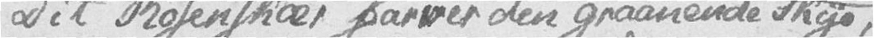Dit Rosenskær farver den graanende Skye,
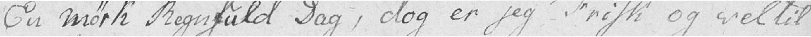En mørk Regnfuld Dag, dog er jeg Frisk og vel til
